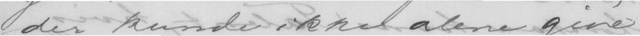der kunde ikke alene give
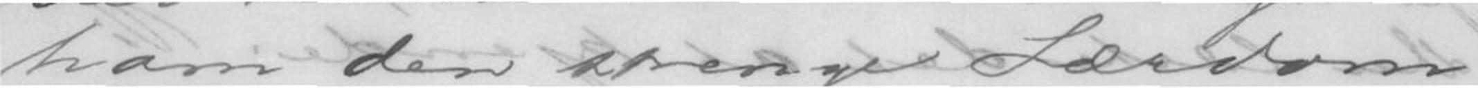ham den Strenge Lærdom
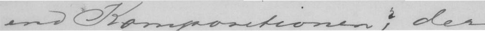end Kompositionen; der
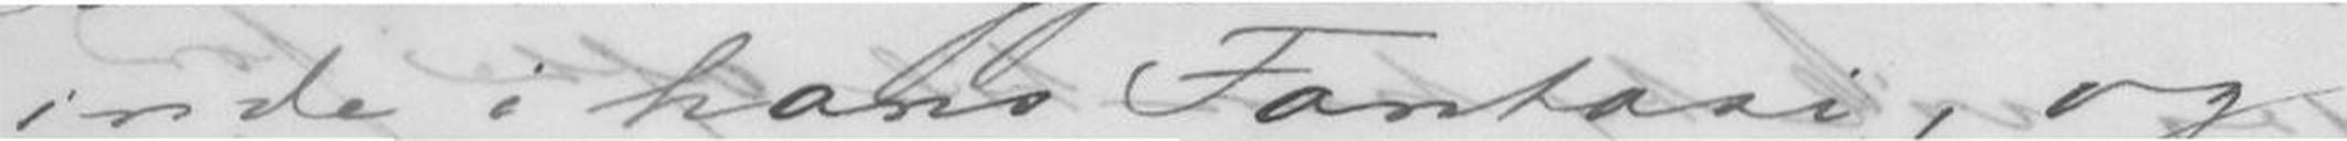inde i hans Fantasi, og
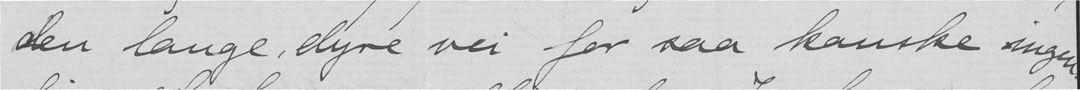den lange, dyre vei for saa kanske ingen-
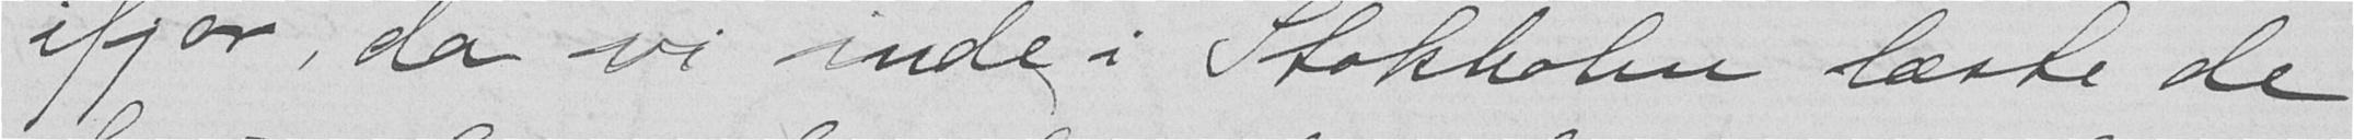ifjor, da vi inde i Stokholm læste de
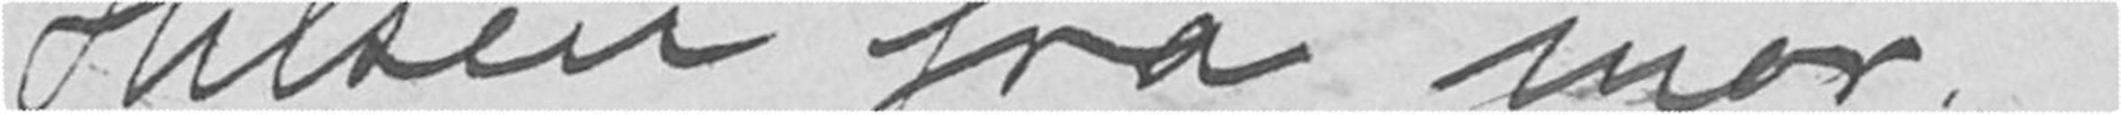Hilsen fra mor!
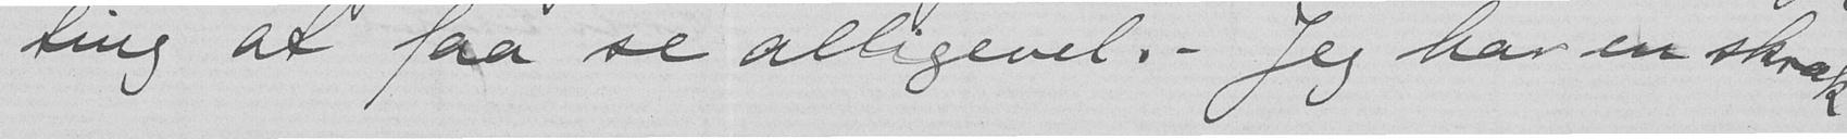ting at faa se alligevel. - Jeg har en skræk
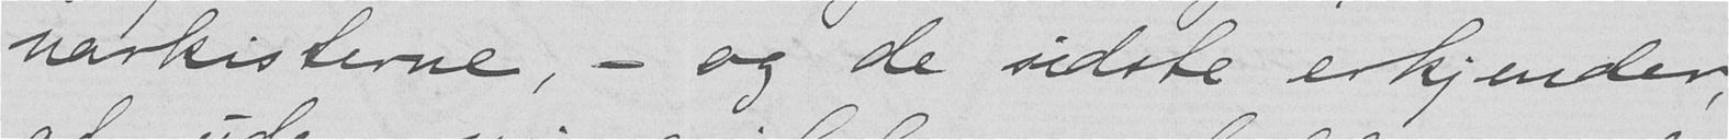narkisterne, - og de sidste erkjender,
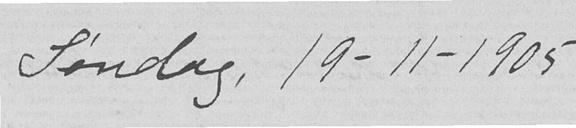Søndag, 19-11-1905
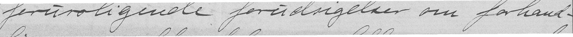foruroligende forudsigelser om forhand-
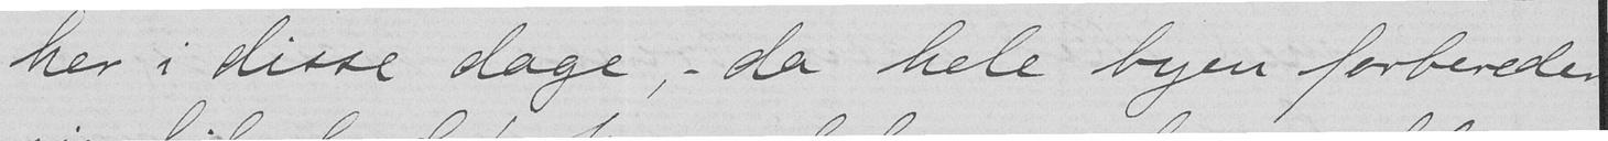her i disse dage, - da hele byen forbereder
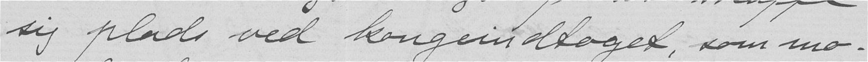sig plads ved kongeindtoget, som mo-
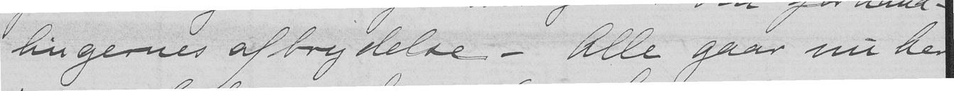lingernes afbrydelse - Alle gaar nu her
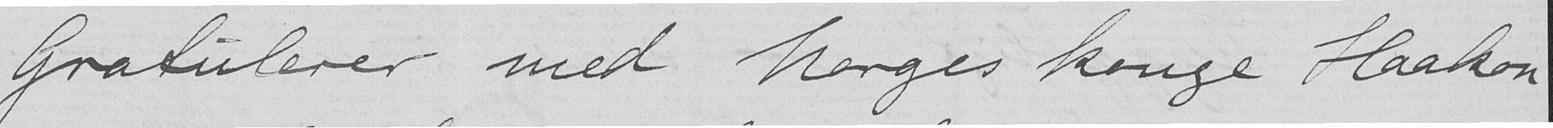Gratulerer med Norges konge Haakon
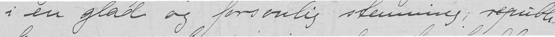i en glad og forsonlig stemning; republi-
